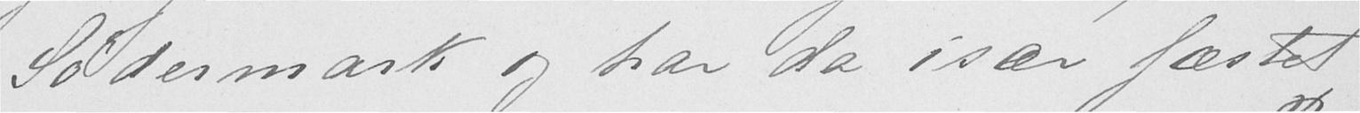Sødermark og har da især fæstet
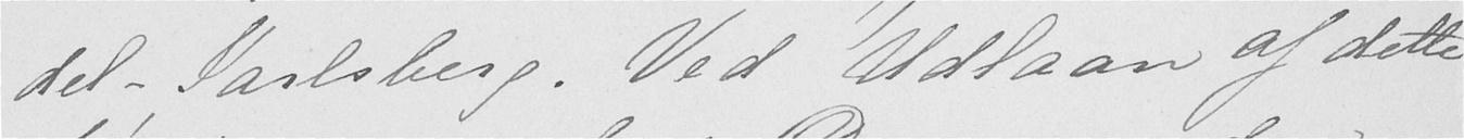del-Jarlsberg. Ved Udlaan af dette
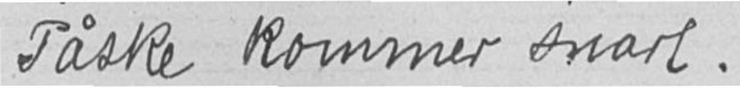Påske kommer snart.
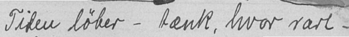Tiden løber - tænk hvor rart -
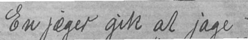En jæger gik at jage
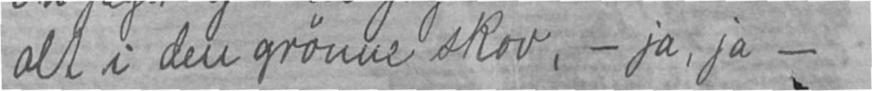alt i den grønne skov - ja, ja -
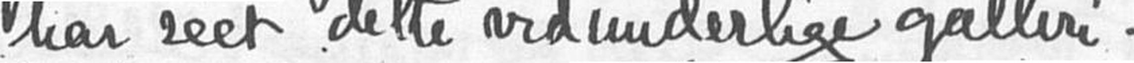har seet dette vidunderlige galleri. -
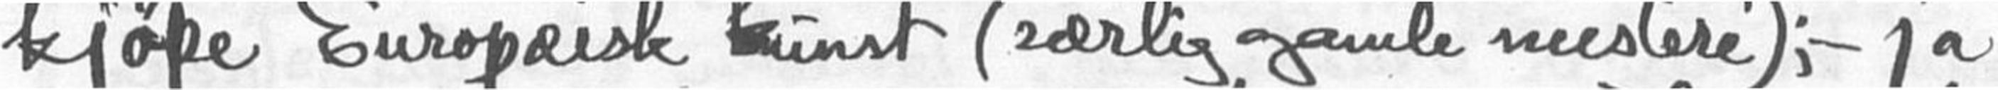kjøpe Europæisk kunst (særlig gamle mestere) : - ja
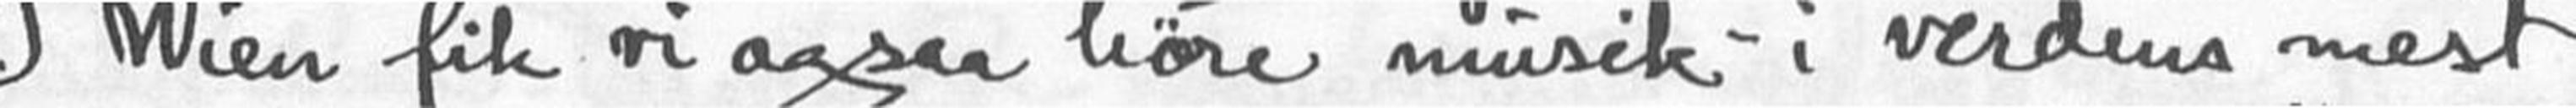I Wien fik vi ogsaa høre musik - i verdens mest
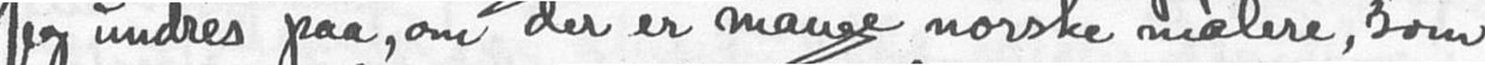Jeg undres paa, om der er mange norske malere, som
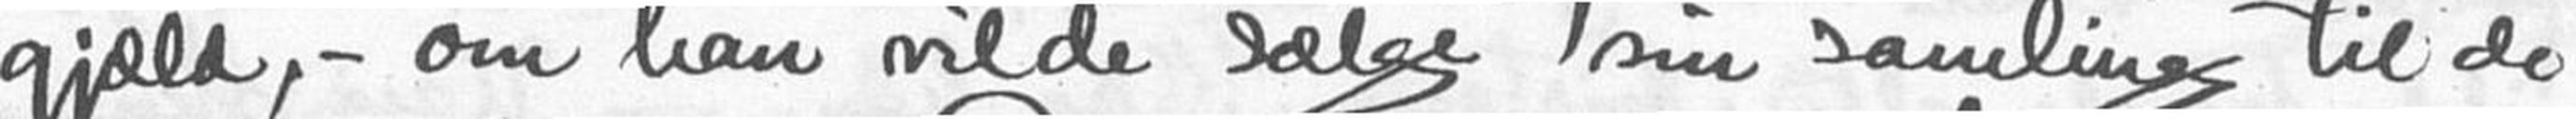gjæld, - om han vilde sælge sin samling til de
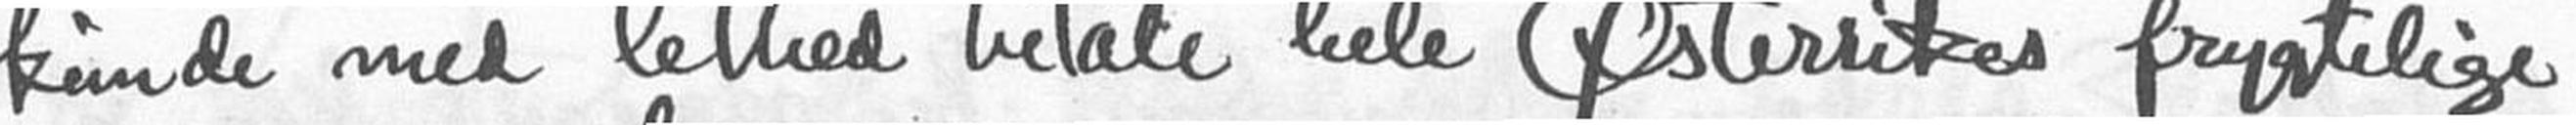kunde med lethed betale hele Østerrikes frygtelige
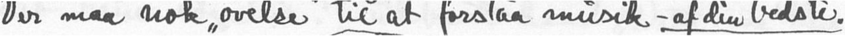Der maa nok "øvelse" til at forstaa musik - af den bedste.
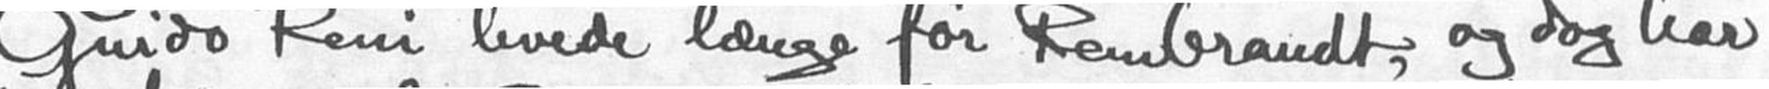Guido Reni levede længe for Rembrandt, og dog har
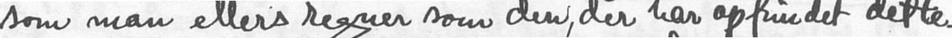som man ellers regner som den, der har opfundet dette.
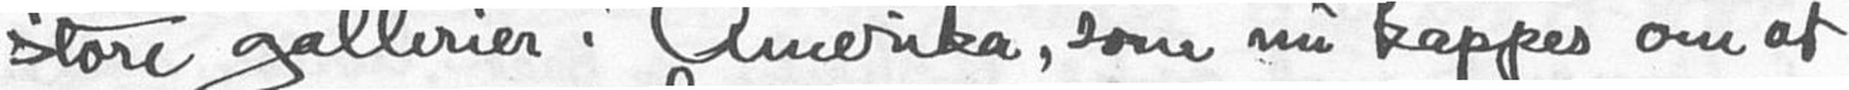store gallerier i Amerika, som nu kappes om at
